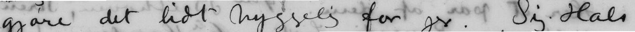gjøre det lidt hyggelig for jer. Sig.Hals
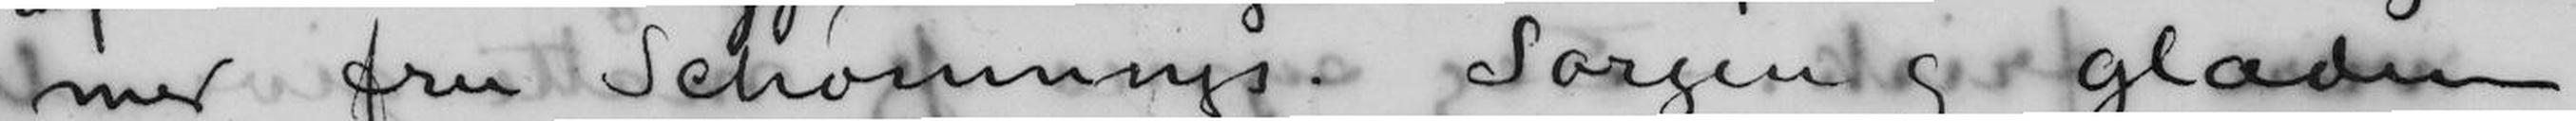med fru Scho...ys. Sorgen og glæden
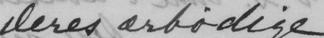Deres ærbødige
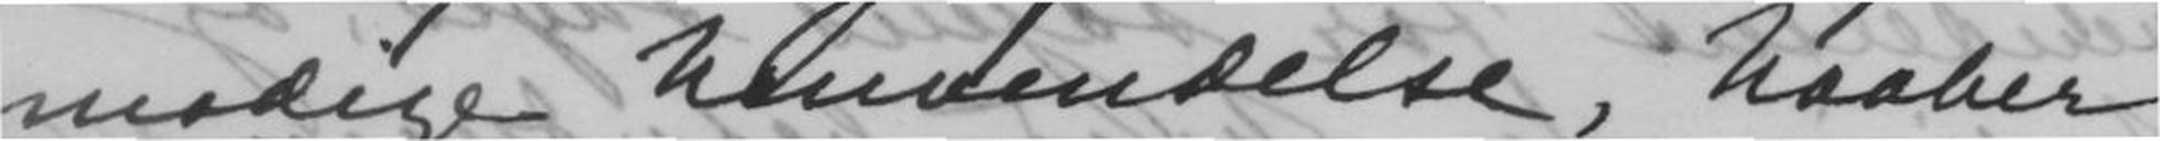modige henvendelse, haaber
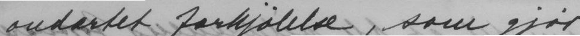ondartet forkjølelse, osm gjør
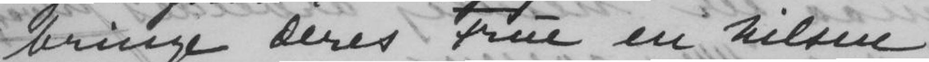bringe Deres Frue en hilsen,
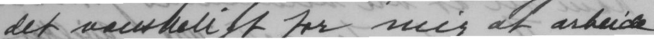det vanskeligt for mig at arbeide
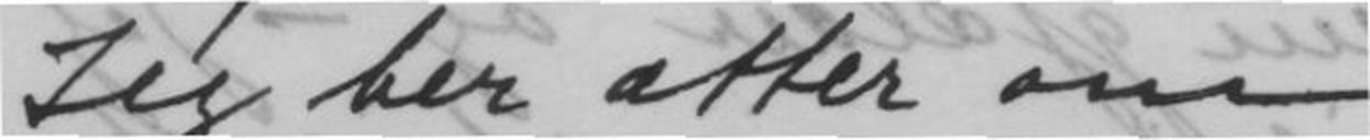Jeg ber atter om
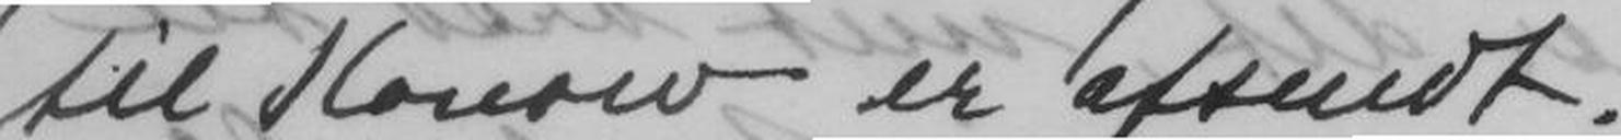til Konow er afsendt.
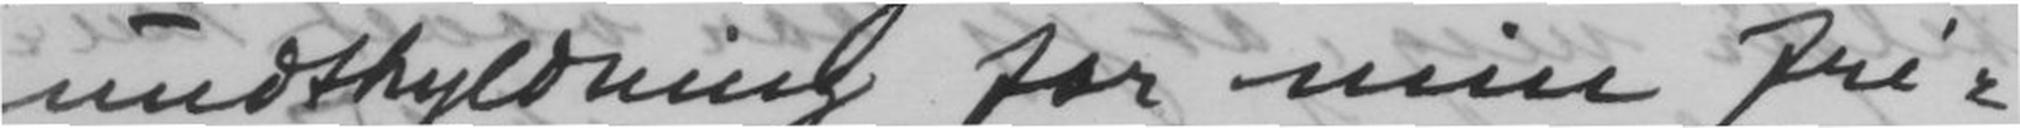undskyldning for min fri-
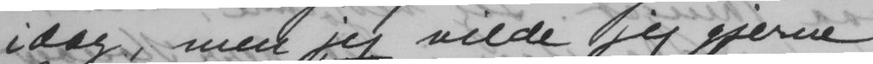idag, men jeg vilde jeg gjerne
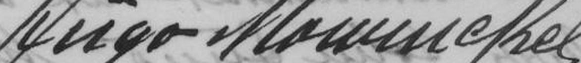Hugo Mowinckel.
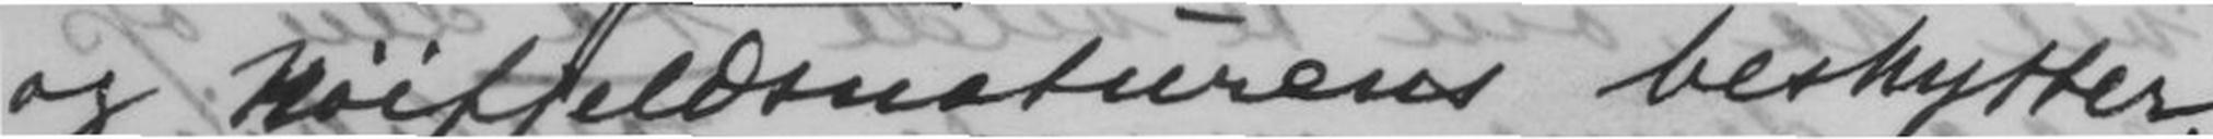og Høifjeldsnaturens beskytter,
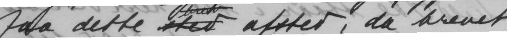faa dette afsted, da brevet
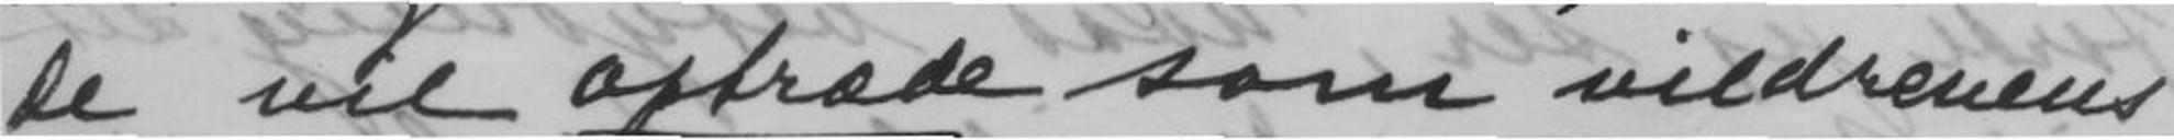De vil optræde som vildrenens
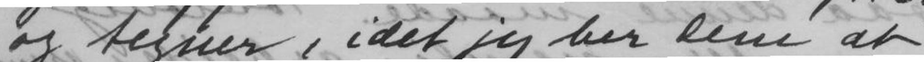og tegner, idet jeg ber dem at
